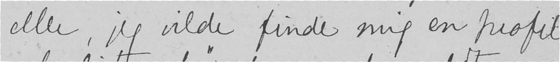eller, jeg vilde finde mig en profil
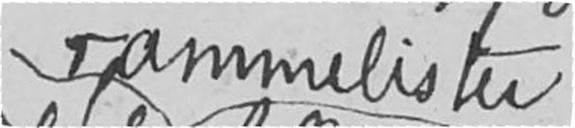rammelister
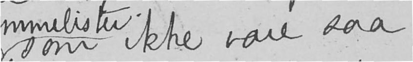som ikke vare saa
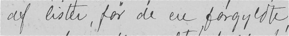af lister, før de ere forgyldte,
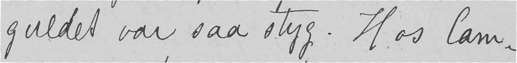guldet var saa styg. Hos Cam-
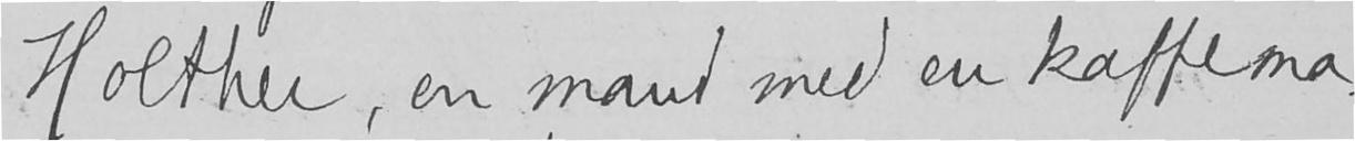Holther, en mand med en kaffema-
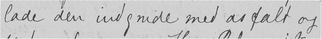lade den indgnide med asfalt og
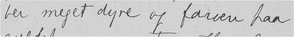ber meget dyre og farven paa
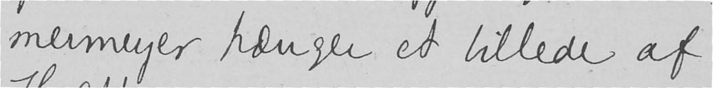mermeyer hænger et billede af
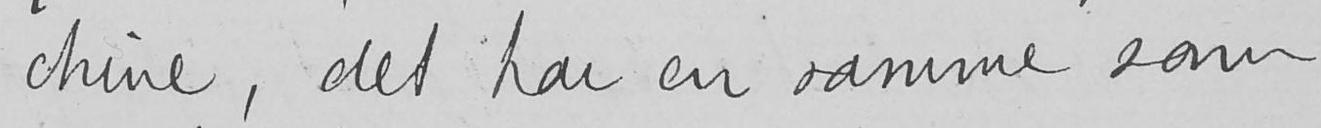chine, det har en ramme som
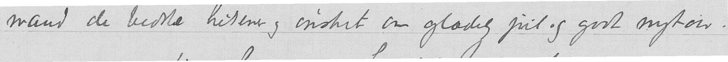mand de bedste hilsener og ønsket om glædelig jul og godt nytaar.
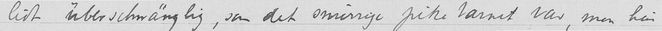lidt überschwänglig, som det snurrige pikebarnet var, men hun
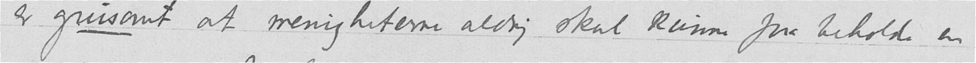er grusomt at menigheterne aldrig skal kunne faa beholde en
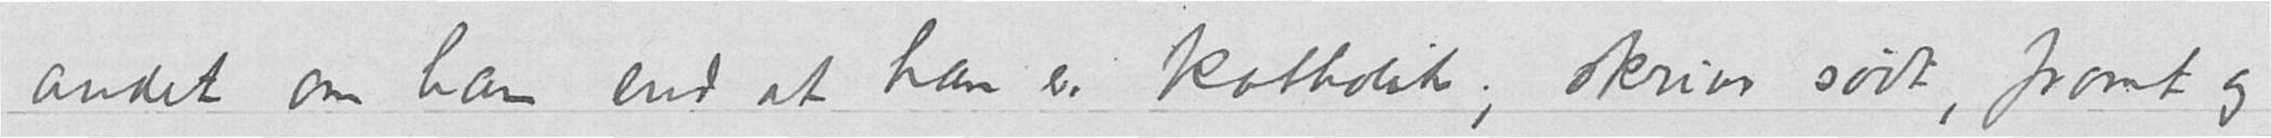andet om ham end at han er katholik; skriver sødt, fromt og
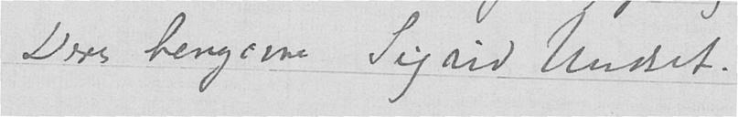Deres hengivne Sigrid Undset.
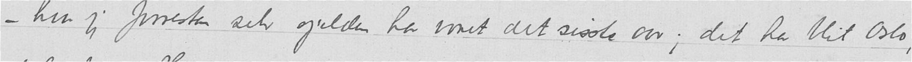– hvor jeg forresten selv sjelden har været det sisste aar; det har blit Oslo,
